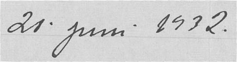20. juni 1932.
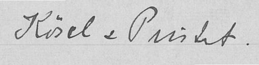Kösel & Pustet.
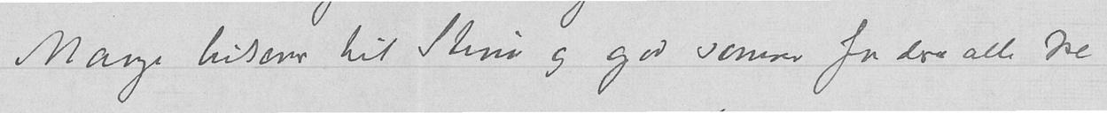Mange hilsener til Stina og god somner for dere alle tre
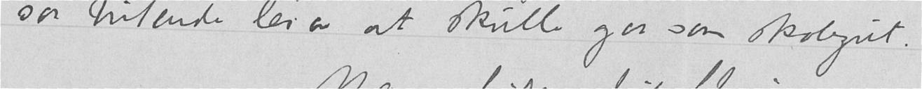saa lutende lei ar at skulle gaa som skolegut.
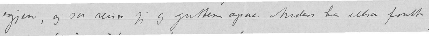igjen, og saa reiser jeg og guttene ogsaa. Anders har altsaa faatt
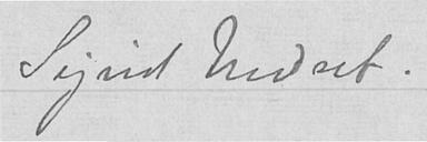Sigrid Undset.
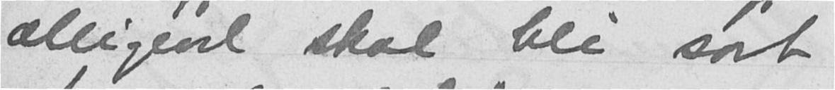alligevel skal bli sort
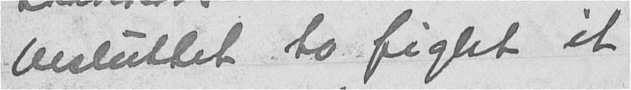besluttet to fight it
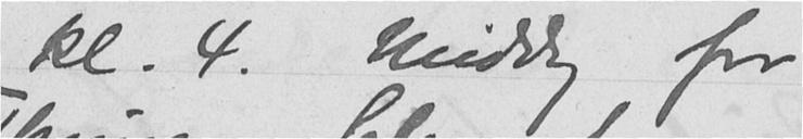kl. 4. Middag for
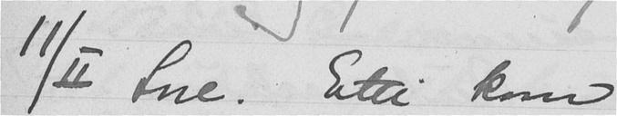11/II Sne. Etti kom
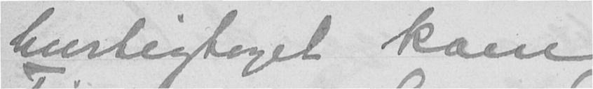hurtigtoget kom
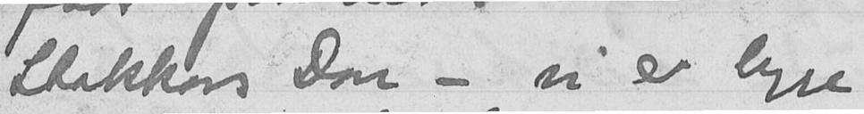Stakkars Don - vi er begge
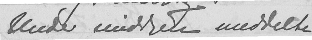Under middagen meddelte
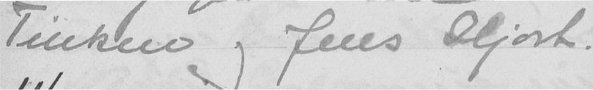Tinken og Jens Hjort.
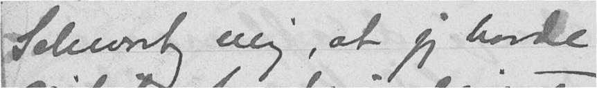Schwartz mig, at jeg havde
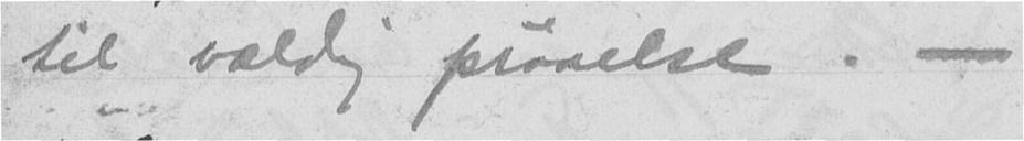til veldig prøvelse. -
